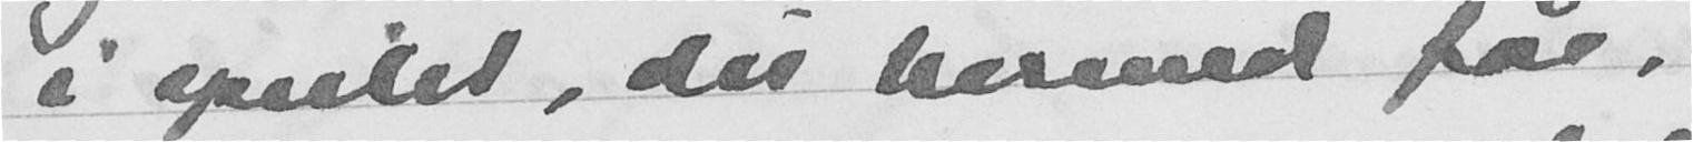i speilet, du hermed får,
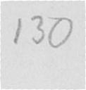130
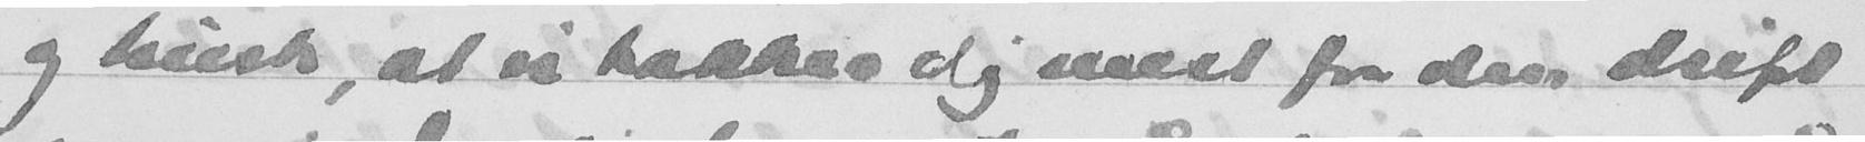og husk, at vi takker dig svært for den drift
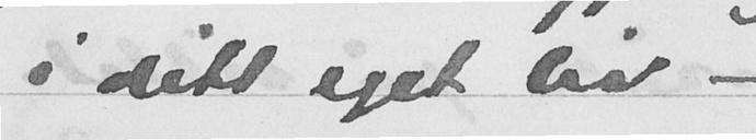i ditt eget liv -
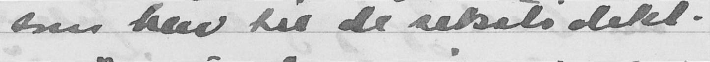som blev til de seksti dikt.
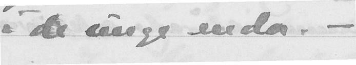i de unge enda. -
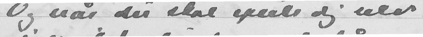Og når du skal speile dig selv
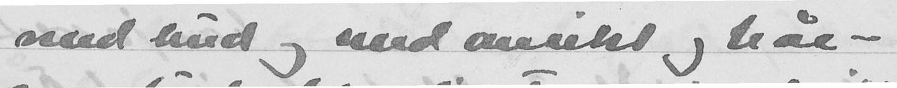med hud og med ansikt og hår -
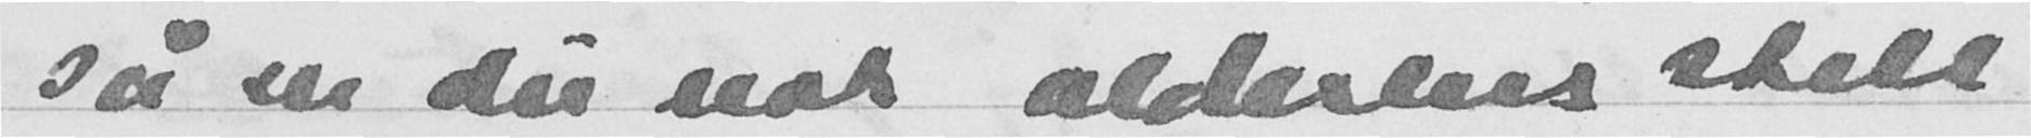så er de nok alderens skall
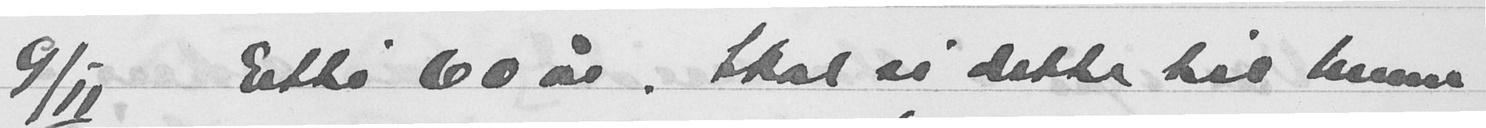9/1 Elli 60 år. Skal si dette til henne
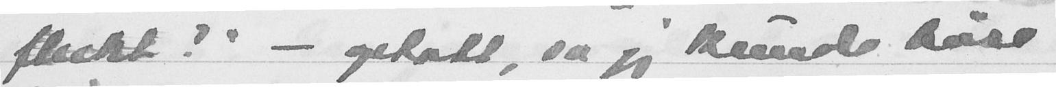flukt!" - opskakt, så jeg kunde høre
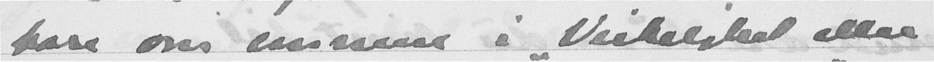bare om emnene i "Virkelighet eller
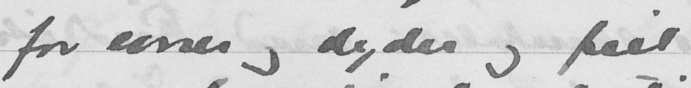for evner og dyder og feil
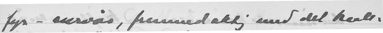fyr - nervøs, fremmedaktig med det kull-
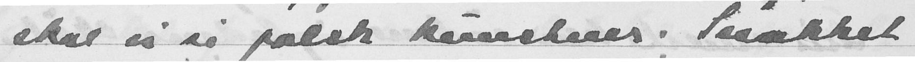skal vi si polsk kunstner. Snakket
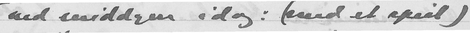ved middgen i dag: (med et speil)
